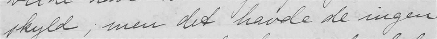skyld; men det havde de ingen
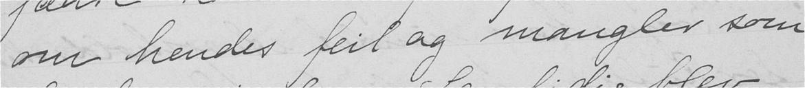om hendes feil og mangler som
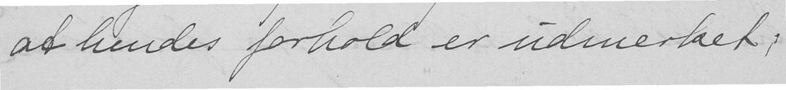at hendes forhold er udmerket,
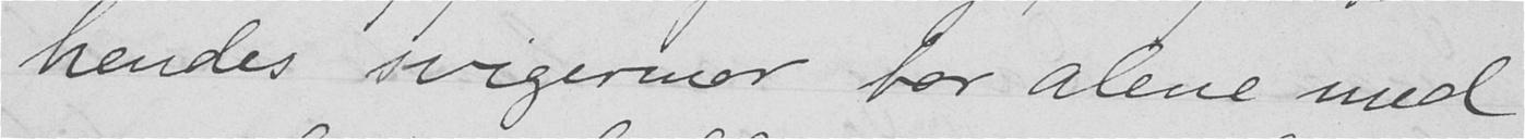hendes svigermor bor alene med
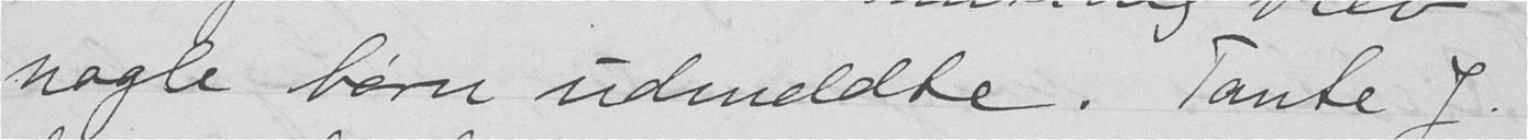nogle børn udmeldte. Tante J.
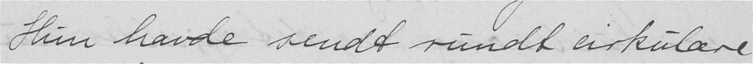Hun havde sendt rundt cirkulære
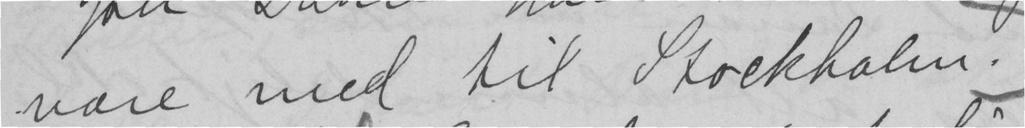være med til Stockholm.
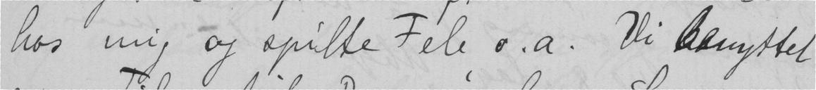hos mig og spilte Fele o. a. Vi benyttet
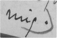mig.
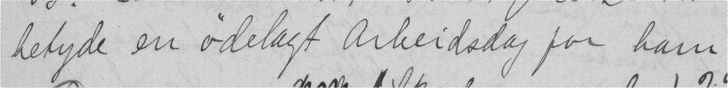betyde en ødelagt Arbeidsdag for ham
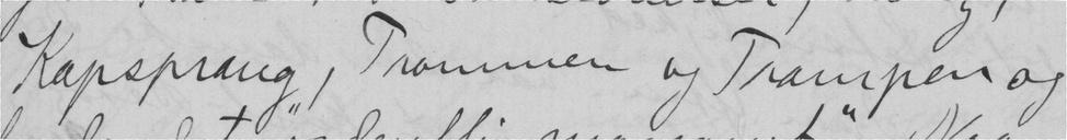Kapsprang, Trommer og Trampen og
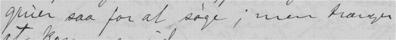gruer saa for at søge; men trænger
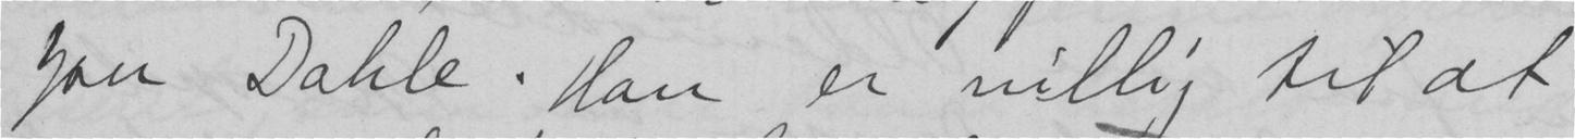Jan Dahle. Han er villig til at
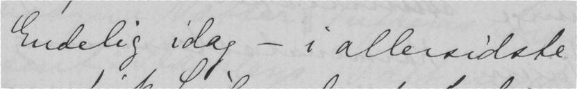Endelig idag - i allersidste
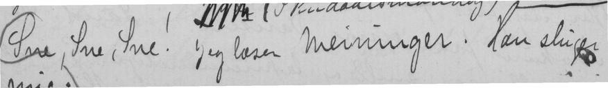Sne, Sne, Sne! Jeg læser Weininger. Han sluger
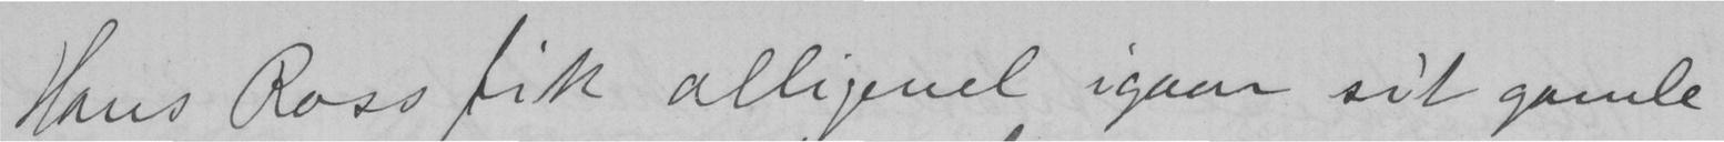Hans Ross fik alligevel igaar sit gamle
